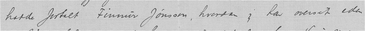hadde fortalt Finnur Jonsson, hvordan jeg har oversat eder
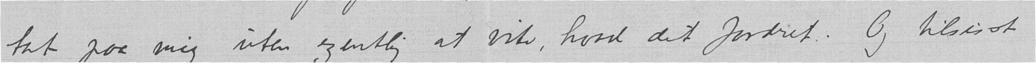tat paa mig uten egentlig at vite, hvad det fordret. Og tilsisst
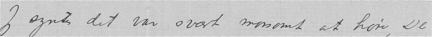Jeg syntes det var svært morsomt at høre, De
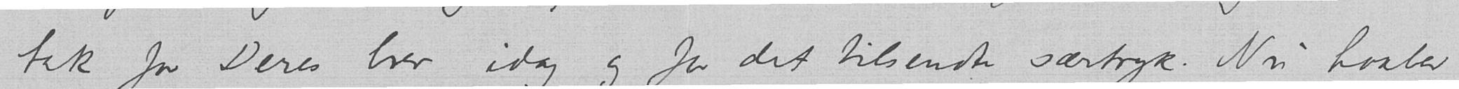tak for Deres brev idag og for det tilsendte særtryk. Nu haaber
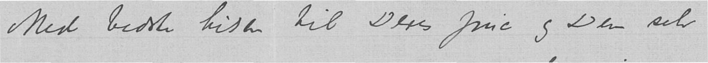Med bedste hilsen til Deres frue og Dem selv
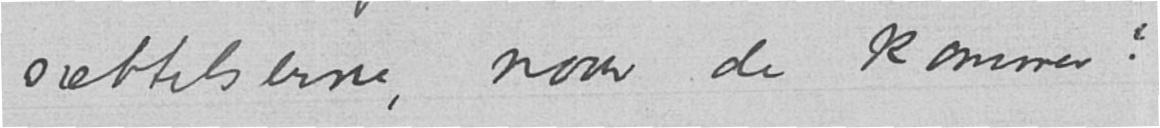sættelserne, naar de kommer?
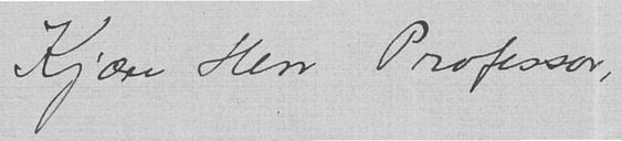Kjære Herr Professor,
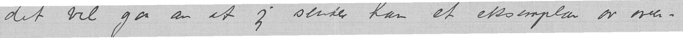det vel gaa an at jeg sender ham et eksemplar av over-
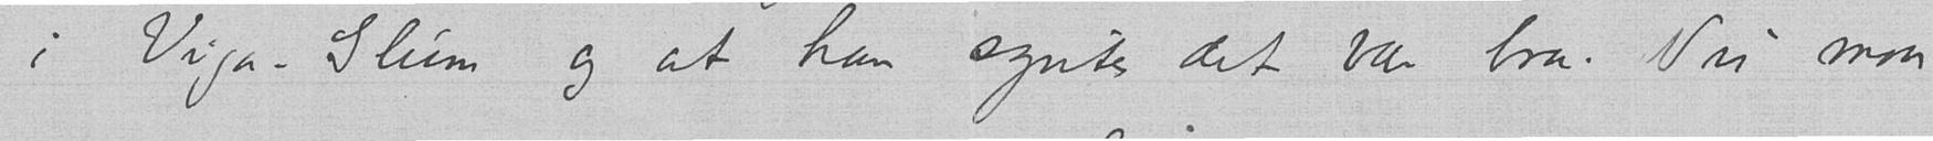i Viga-Glum og at han syntes det var bra. Nu maa
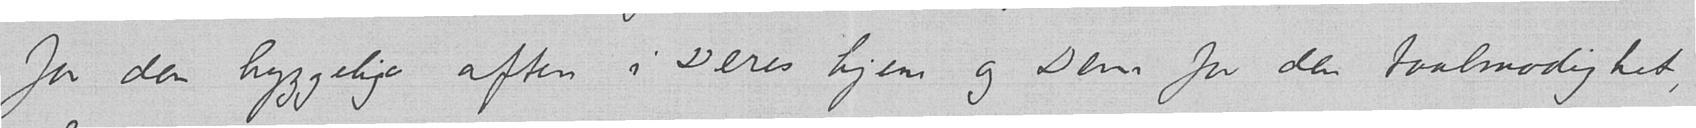for den hyggelige aften i Deres hjem og Dem for den taalmodighet,
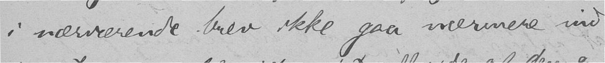i nærværende brev ikke gaa nærmere ind
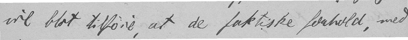vil blot tilføie, at de faktiske forhold, med
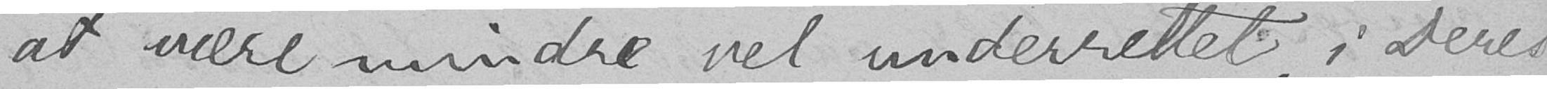at være mindre vel underrettet, i Deres
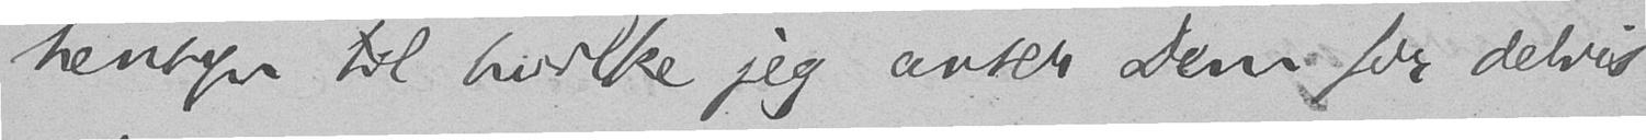hensyn til hvilke jeg anser Dem for delvis
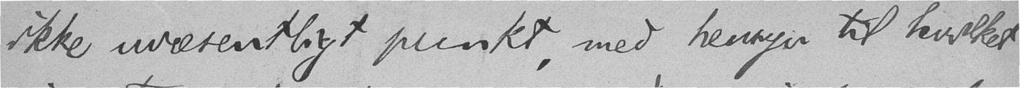ikke uvæsentligt punkt, med hensyn til hvilket
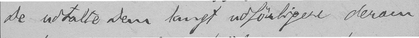De udtalte Dem langt udførligere derom
